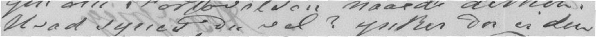Hvad synes Du vel? ynker Du ei den
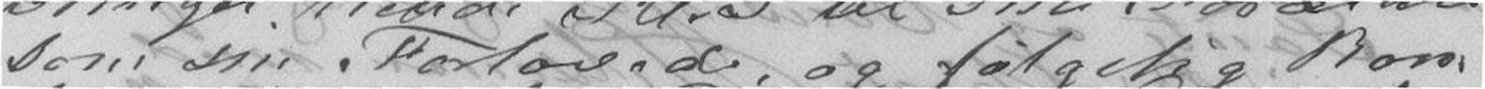som sin Forlovede, og følgelig kom
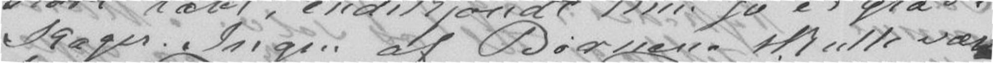Kager. Ingen af Børnene skulle være
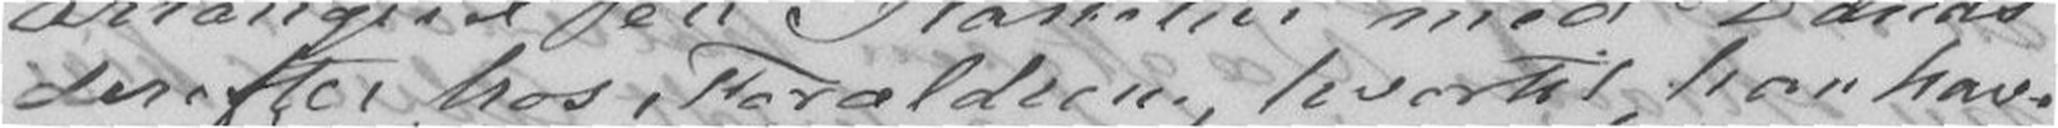derefter hos Forældrene, hvortil han hav-
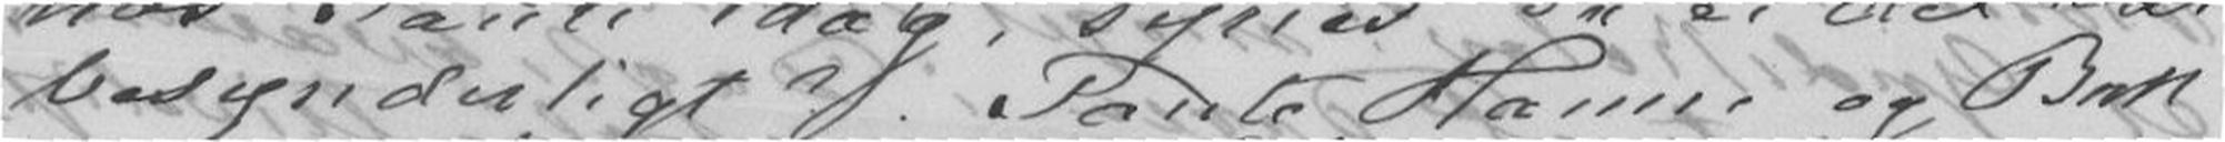besynderligt ? Tante Hanne og Bull
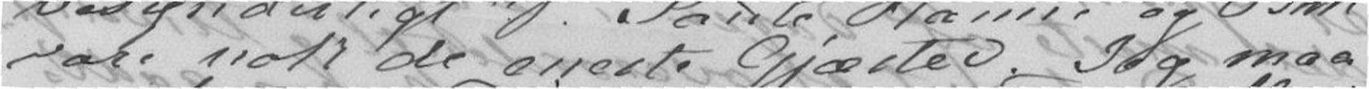vare nok de eneste Gjæster. Jeg maa
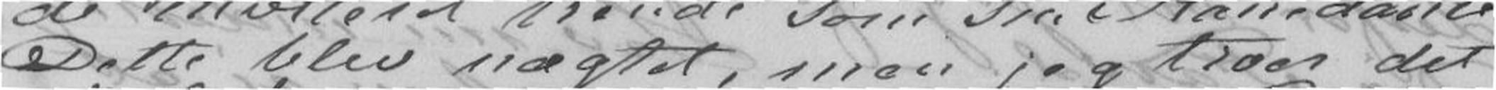Dette blev nægtet, men jeg troer det
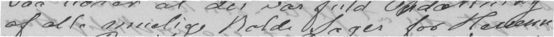af alle muelige kolde Sager for Herrerne
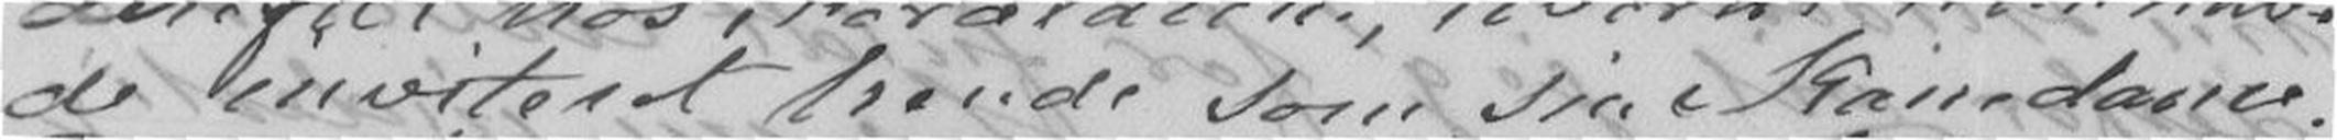de inviteret hende som sin Kanedame.
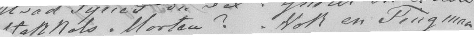stakkels Morten? Nok en Ting maa
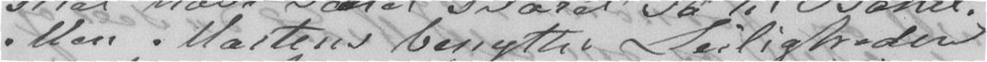Men Martens benytter Leiligheden
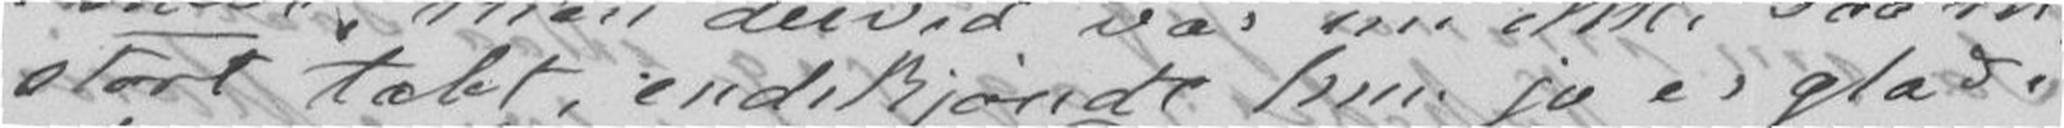stort tabt, endskjøndt hun jo er glad i
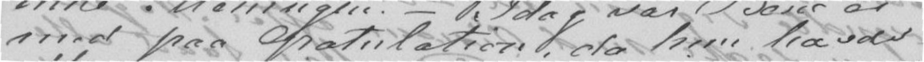med paa Gratulatiom, da hun havde
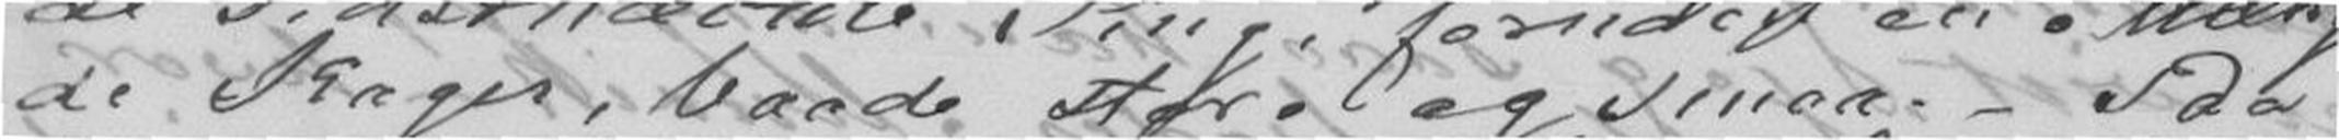de Kager, baade store og smaa. - Paa
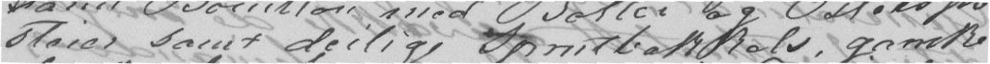steier samt deilige Sprutbakkels, ganske
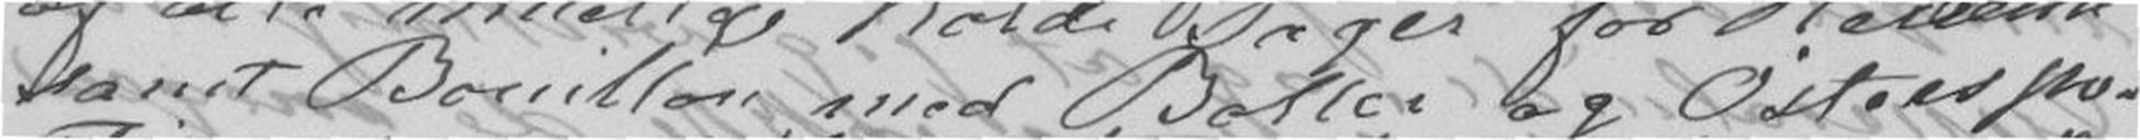samt Bouillon med Boller og Østers po-
